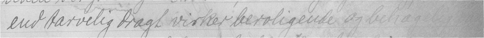end tarvelig dragt virker beroligende og behagelig paa
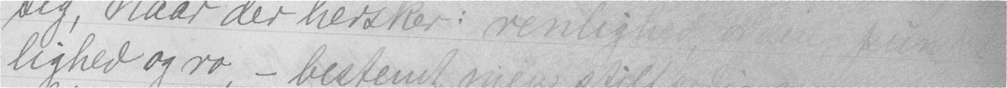sig, naar der hersker: renlighed, , punkte-
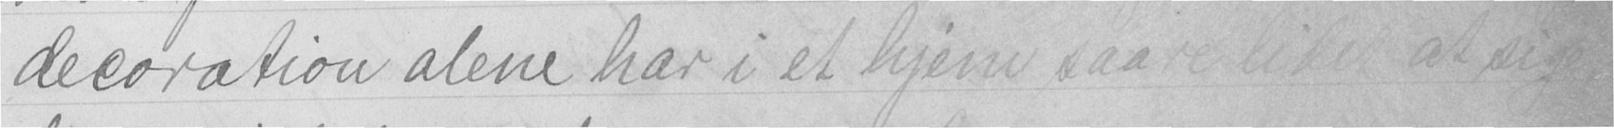decoration alene har i et hjem saare lidet at sige,
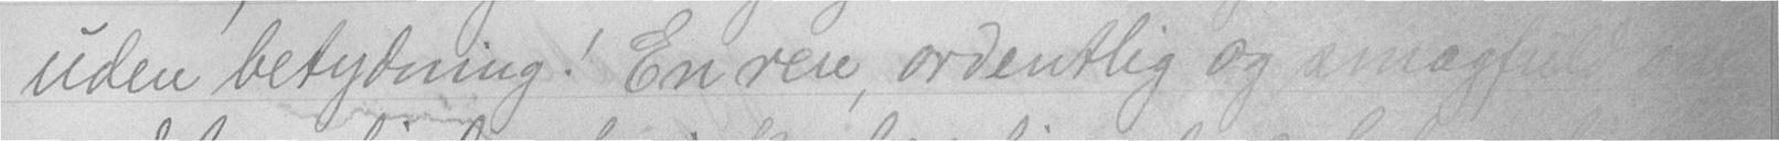uden betydning! En ren, ordentlig og smagfuld
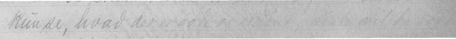kun se, hvad der er godt og skjønt, dette vil da
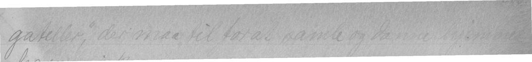gateller," der maa til for at samle og danne hjemmet
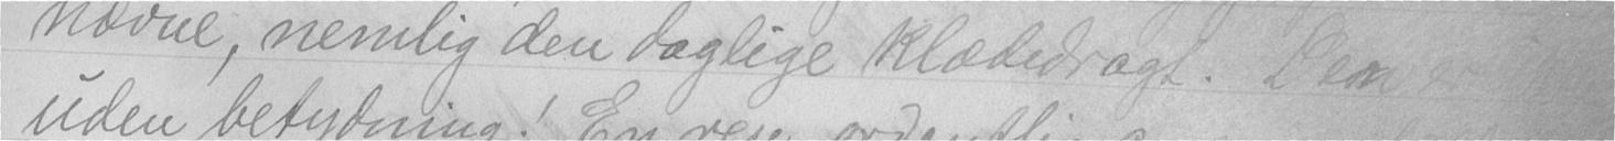nævne, nemlig den daglige klædedragt. Den er ikke
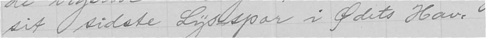sit sidste Lys-spor i Ødets Hav.
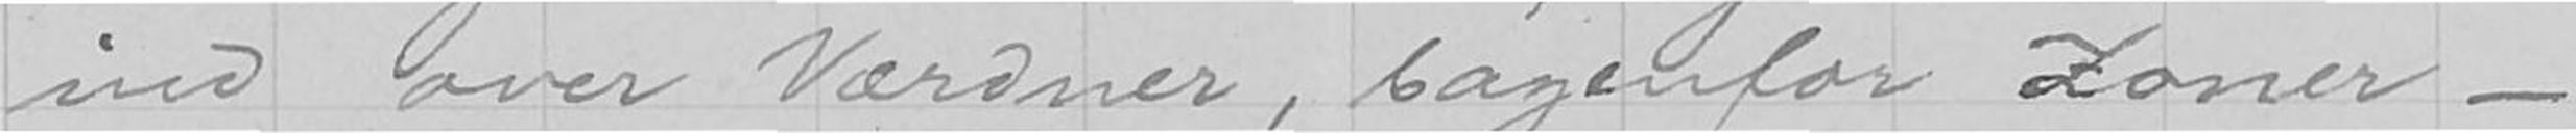ind over Værdner, bagenfor Zoner -
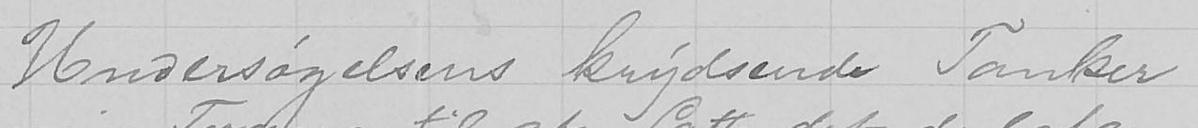Undersøgelsens krydsende Tanker
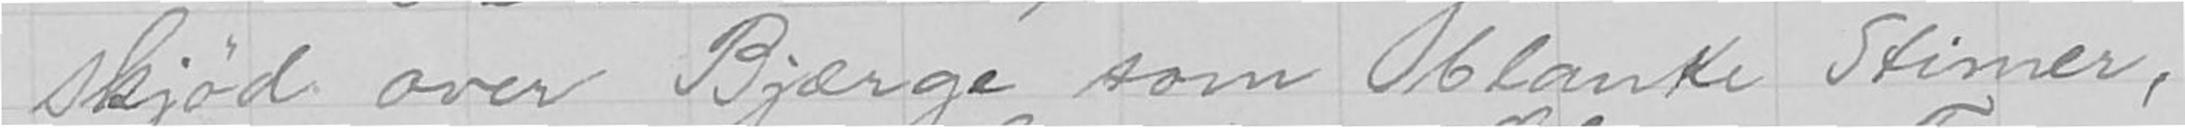skjød over Bjærge som blanke Stimer,
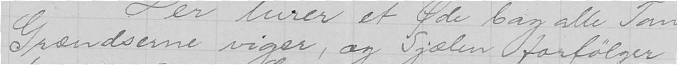Grændserne viger, og Sjælen forfølger
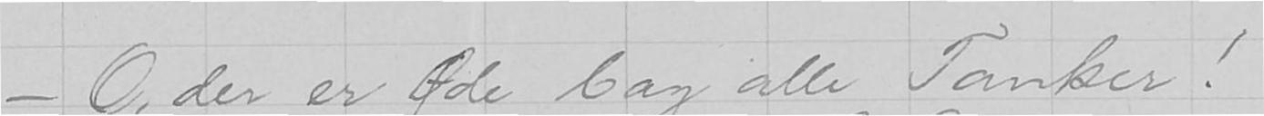- O, der er Øde bag alle Tanker!
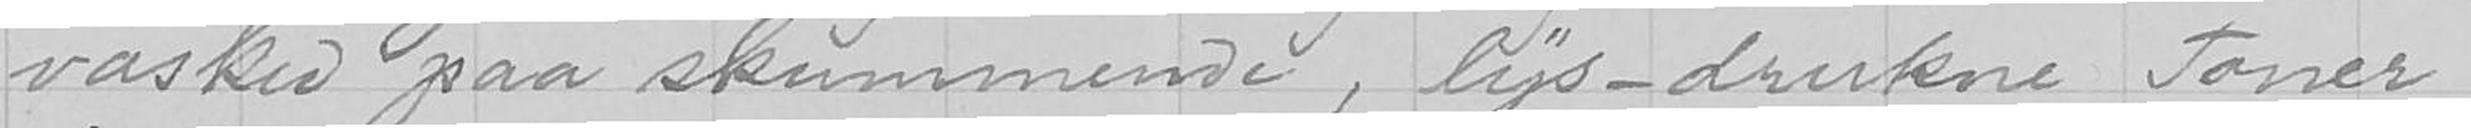vasked paa skummende, lys-drukne Toner
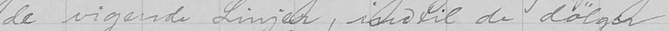de vigende Linjer, indtil de dølger
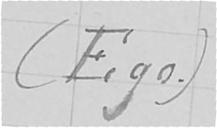(Ego.)
